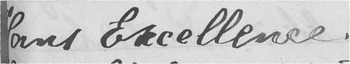Hans Excellence.
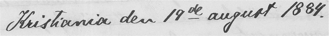Kristiania den 19de august 1884.
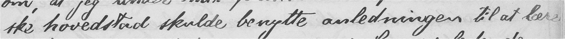ske hovedstad skulde benytte anledningen til at lære
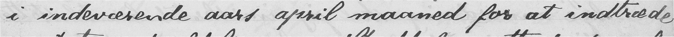i indeværende aars april maaned for at indtræde
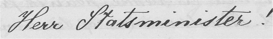Herr Statsminister!
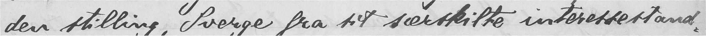den stilling, Sverge fra sit særskilte interessestand-
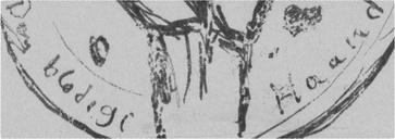Den blodige Haand
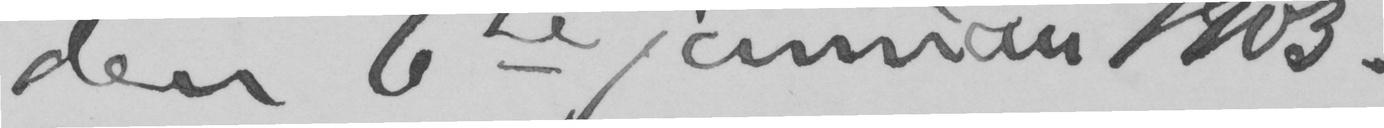den 6te januar 1903.
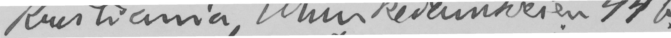Kristiania, Munkedamsveien 44 b.,
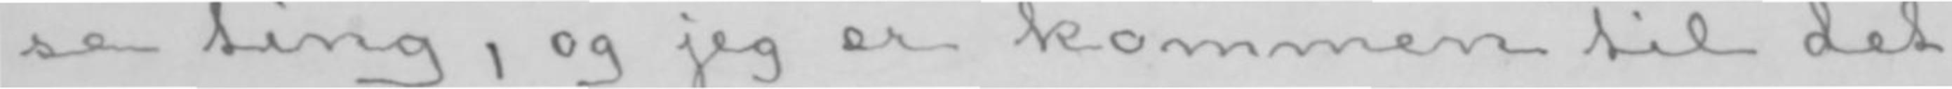se ting, og jeg er kommen til det
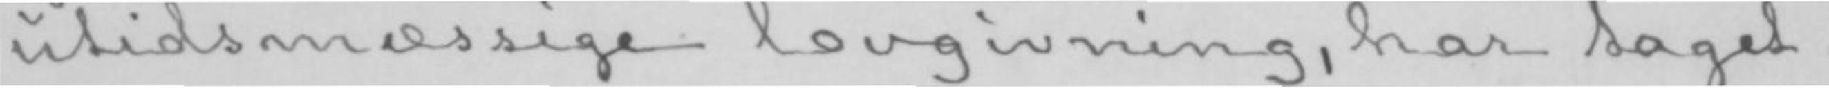utidsmæssige lovgivning, har taget.
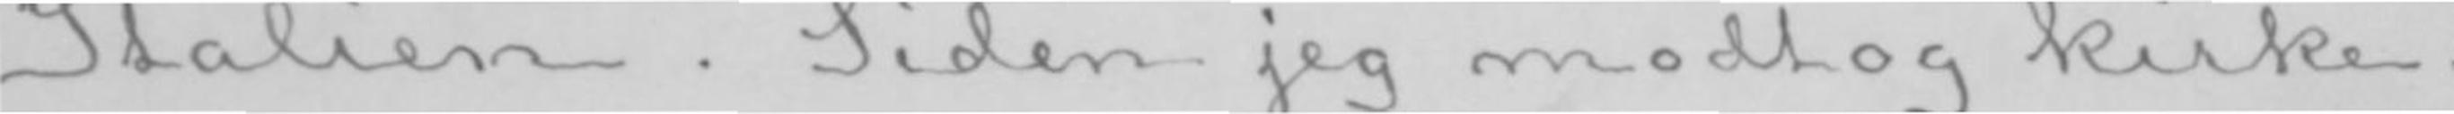Italien. Siden jeg modtog kirke-
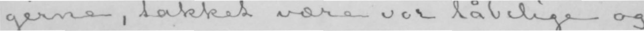gerne, takket være vor tåbelige og
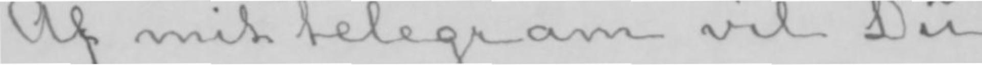Af mit telegram vil Du
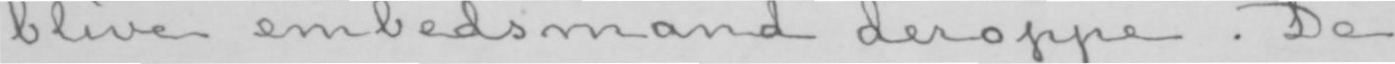blive embedsmand deroppe. De
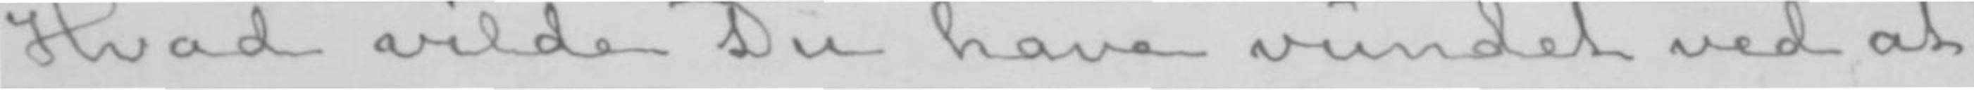Hvad vilde Du have vundet ved at
